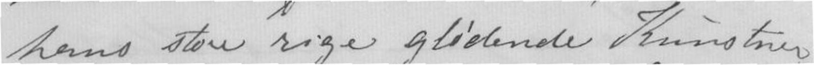hans store rige glødende Kunstner-
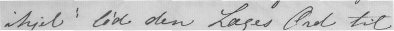ihjel lød den Læges Ord til
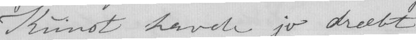Kunst havde jo dræbt
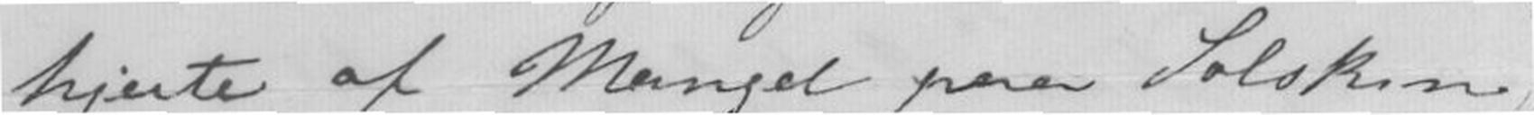hjerte af Mangel paa Solskin,
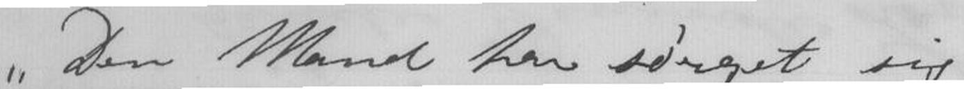Den Mand har sørget sig
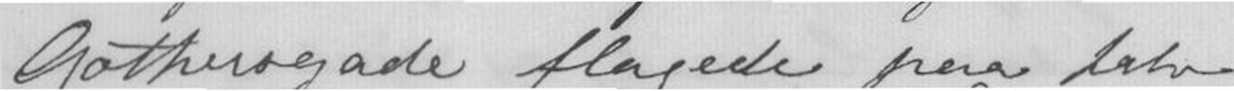Gothersgade flagede paa halv
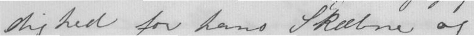dighed for hans Skæbne og
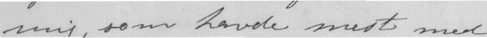mig, som havde mest med
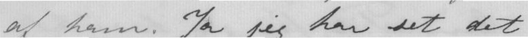af ham. Ja jeg har set det
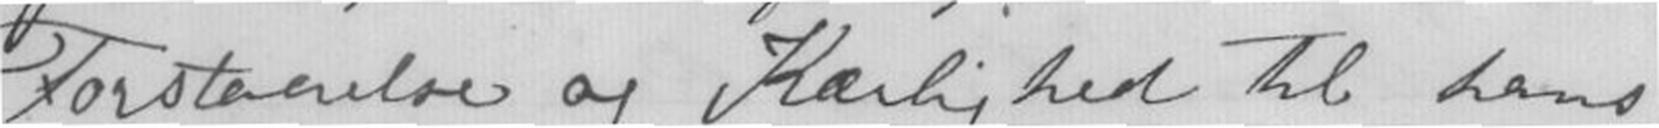Forstaaelse og Kærlighed til hans
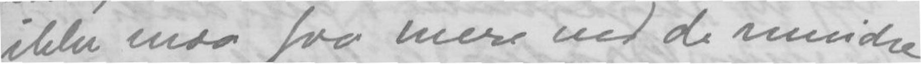ikke maa fa mere end de mindre
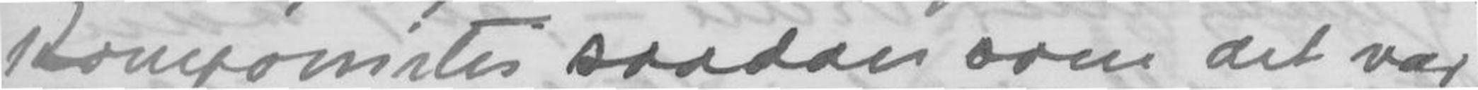Komponister saadan som det var
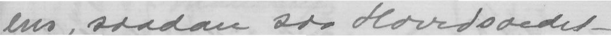ens, saadan saa Hovedsaedet
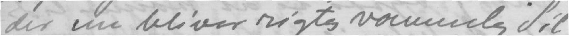der nu bliver rigtig vaemmelig Sil-
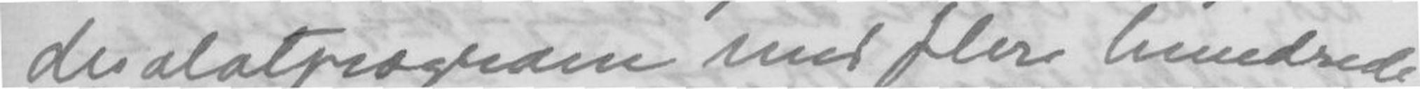desalatprogram med flere hundrede
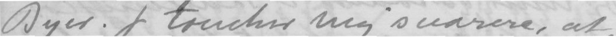Byer. J taenker mig snarere, at
